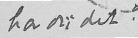har du det?
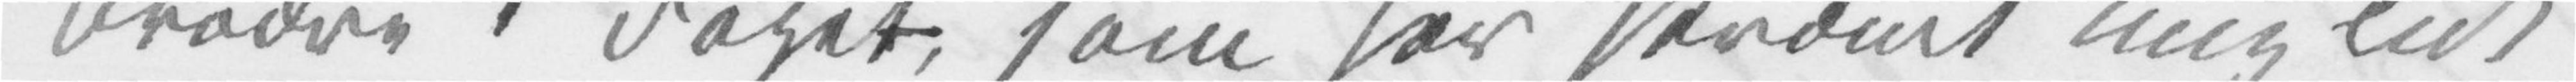Brødre i Faget, som har skræmt mig lidt
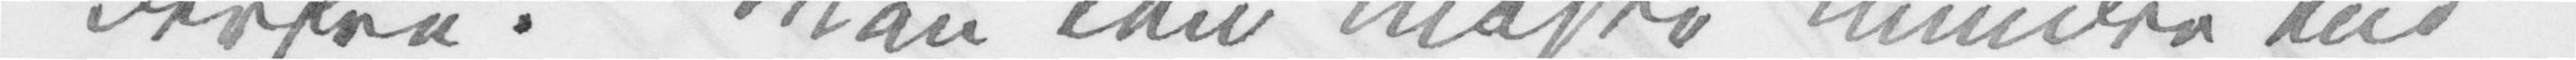derfra. Man kan måske mindre end
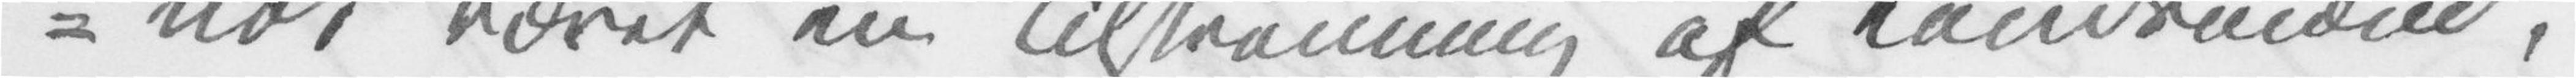nok været en Tilstrømning af Landsmænd,
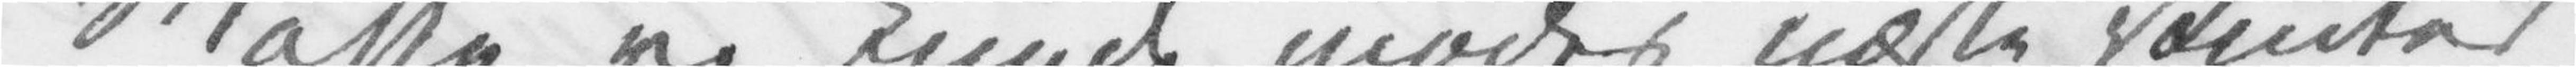Måske vi kunde mødes næste Vinter
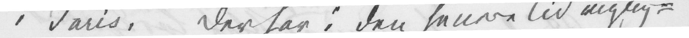i Paris? Der har i den senere Tid rigtig=
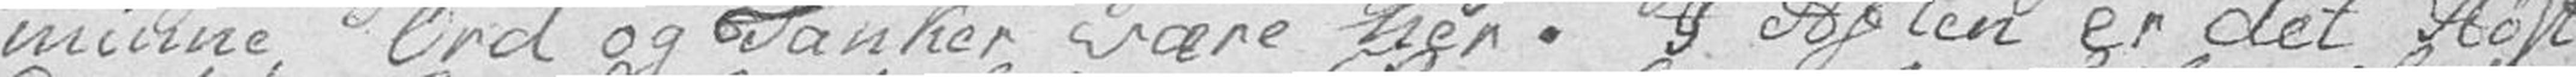minne Ord og Tanker være her. I Aften er det Høst
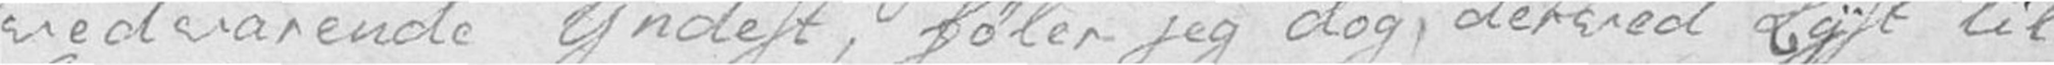vedvarende yndest, føler jeg dog, derved Lyst til
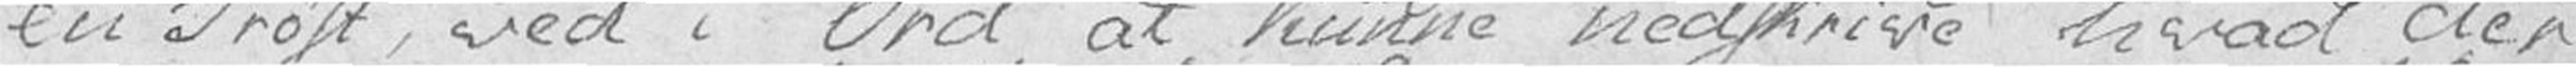en Trøst, ved i Ord at kunne nedskrive hvad der
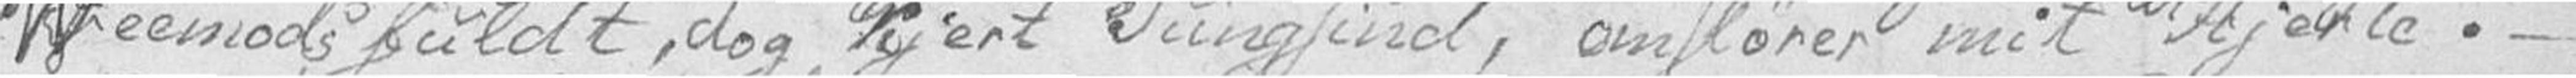Veemodsfuldt, dog kjert Tungsind, omslører mit Hjerte. –
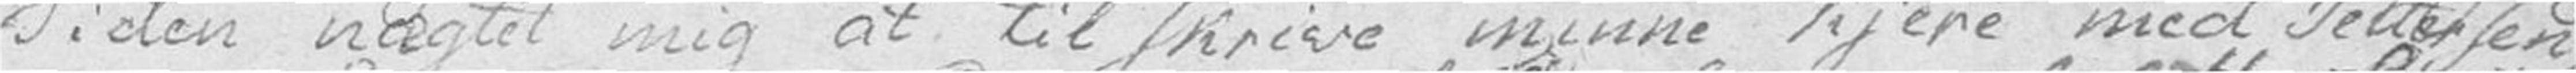Tiden nægtet mig at tilskrive minne kjere med Pettersen,
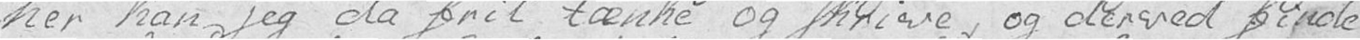her kan jeg da frit tænke og skrive, og derved finde
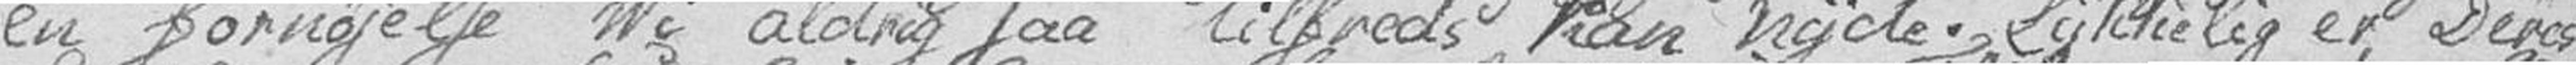en fornøjelse Vi aldrig saa tilfreds kan nyde. Lykkelig er Deres
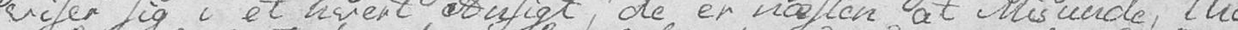viser sig i et hvert Ansigt, de er næsten at Misunde, thi
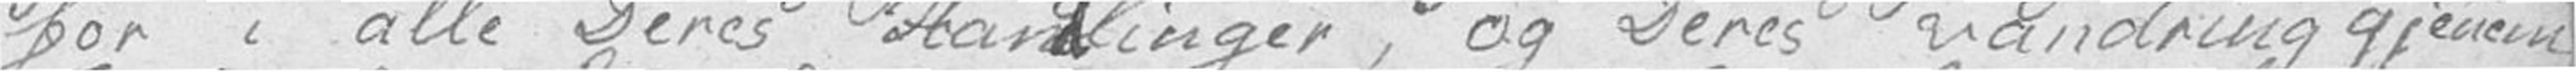for i alle Deres Handlinger, og Deres vandring gjennem
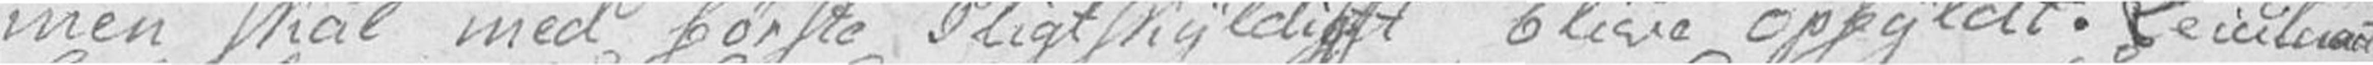men skal med første Pligtskyldighet blive opfyldt. Leiutenant
